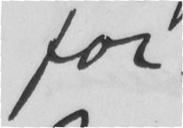for
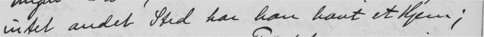intet andet Sted har han havt et Hjem;
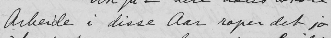Arbeide i disse Aar røper det jo
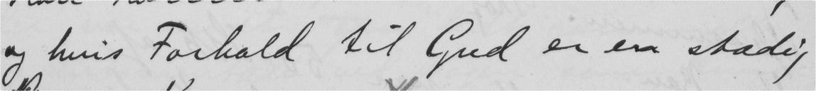og hvis Forhold til Gud er en stadig
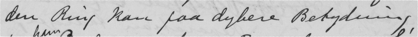den Ring kan faa dybere Betydning
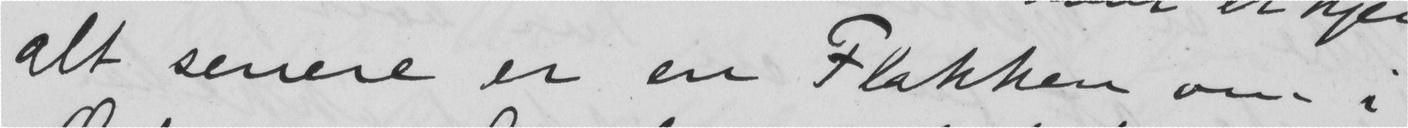alt senere er en Flakken om i
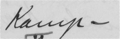Kamp -
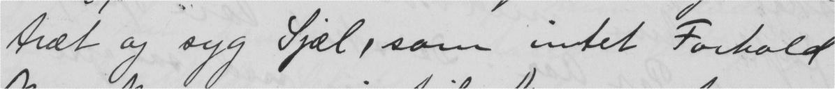træt og syg Sjæl, som intet Forhold
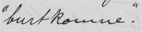"bortkomne."
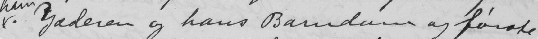. Jaderen og hans Barndom og første
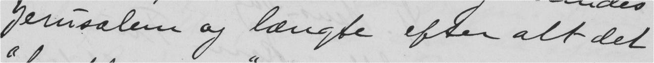Jerusalem og længte efter alt det
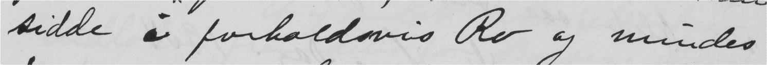sidde i forholdsvis Ro og mindes
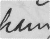ham
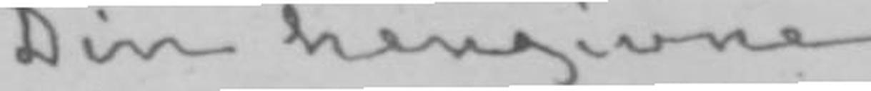Din hengivne
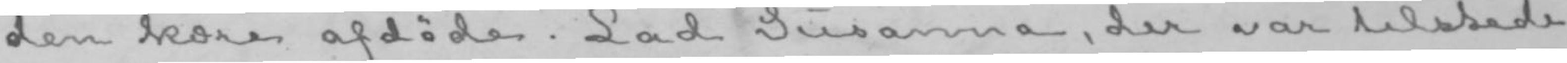den kære afdøde. Lad Susanna, der var tilstede,
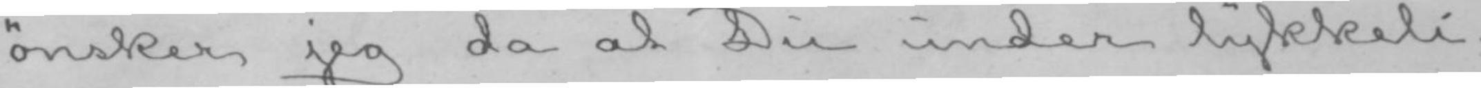ønsker jeg da at Du under lykkeli-
